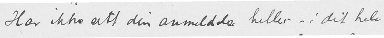Har ikke sett din anmeldelse heller  – i det hele
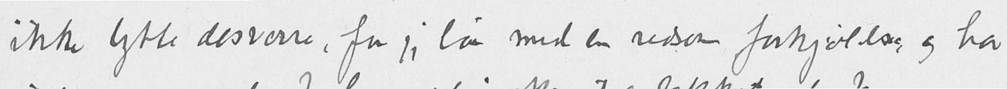ikke lytte desverre, for jeg laa med en redsom forkjølelse, og har
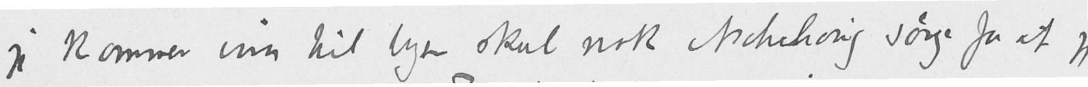jeg kommer inn til byen skal nok Aschehoug sørge for at jeg
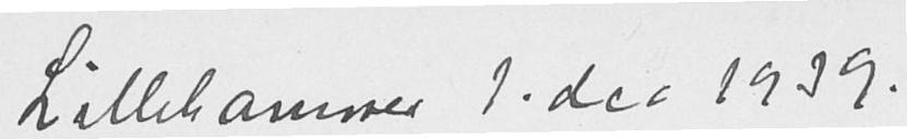Lillehammer 1.dec. 1939.
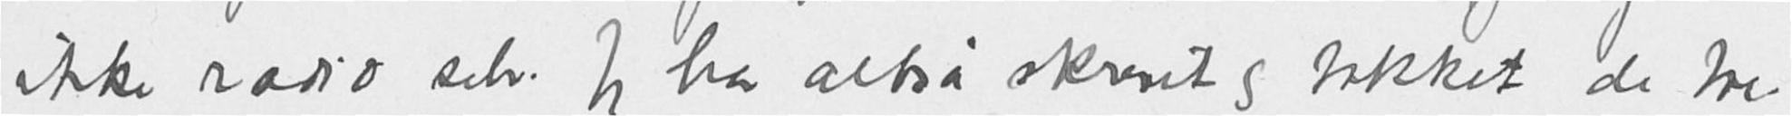ikke radio selv. Jeg har altsa skrevet og takket de tre.
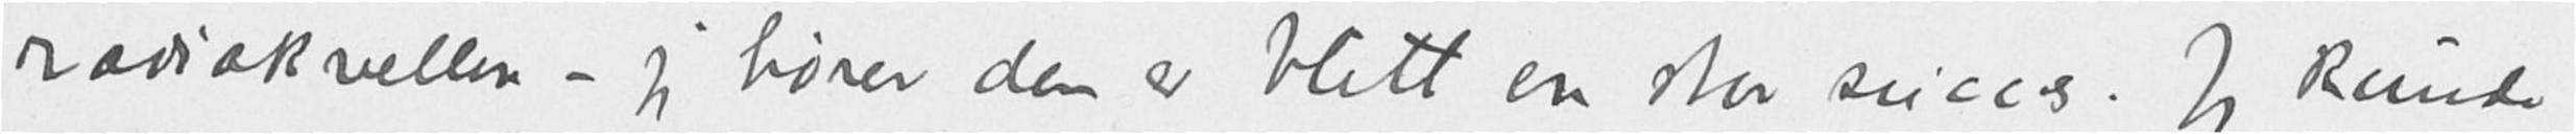radiokvellen – jeg hører den er blitt en stor succes. Jeg kunde
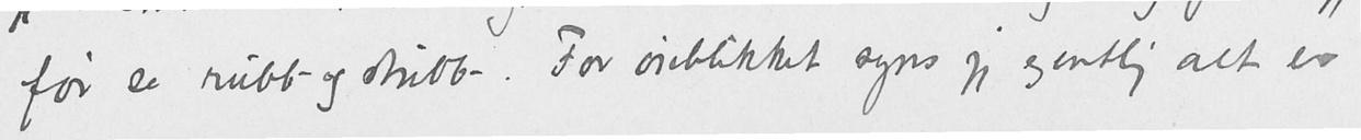faar se rubb- og stutt –. For øieblikket syns jeg egentlig alt er
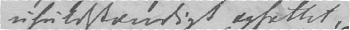ufuldstændigt opfattet,
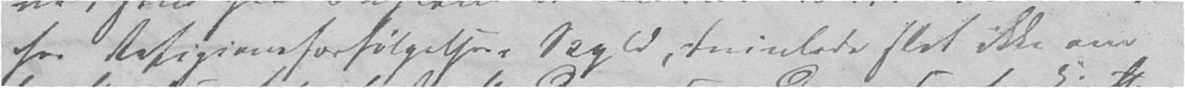for Religionsforfølgelsens Skyld, tvivlede slet ikke om
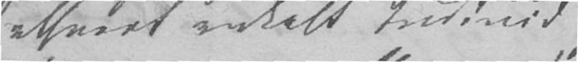ethvert enkelt Individ
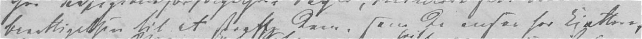Berettigelsen til at straffe dem, som de ansaa for Kjettere,
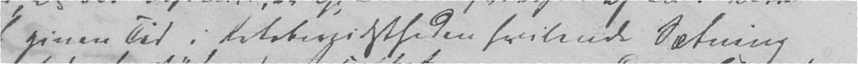given Tid i Retsbevidstheden hvilende Sætning
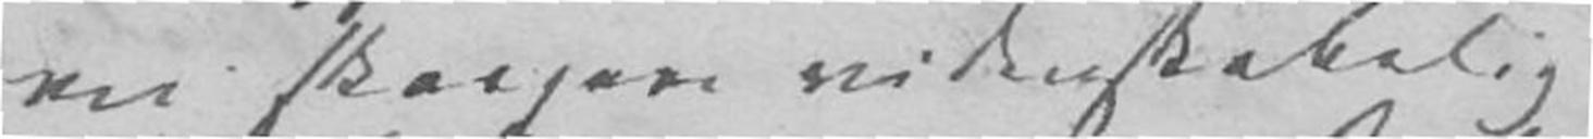en skarpere videnskabelig
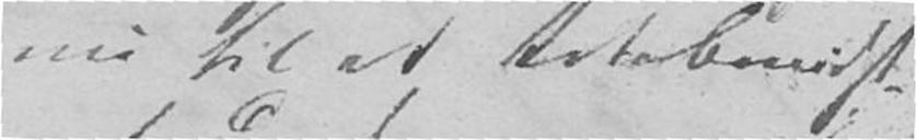nu til at Retsbevidst-
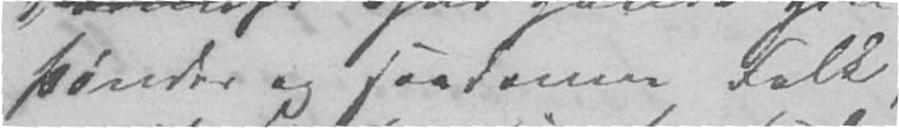Bønder og saadanne Folk
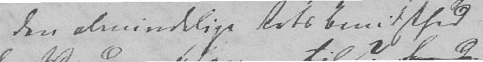den almindelige Retsbevidsthed
